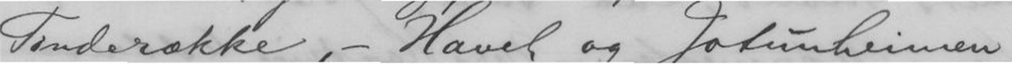Tinderække, - Havet og Jotunheimen
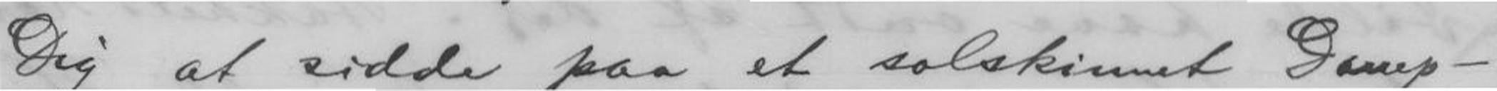Dig at sidde paa et solskinnet Damp-
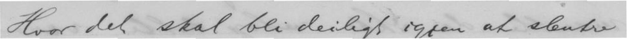Hvor det skal bli deiligt igjen at slentre
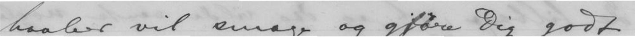haaber vil smage og gjøre Dig godt.
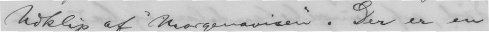Udklipp af "Morgenavisen". Der er en
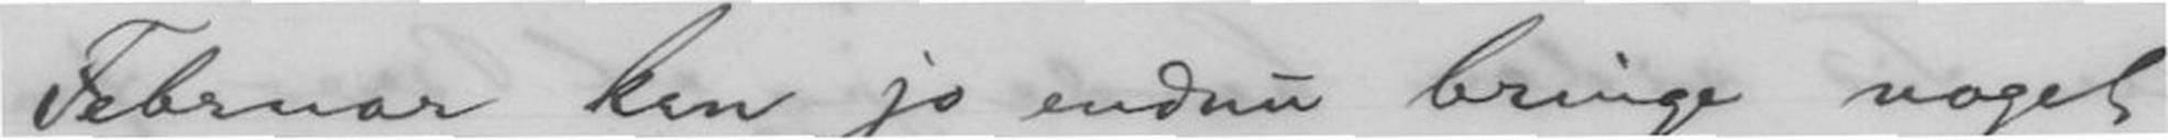Februar kan jo endnu bringe noget
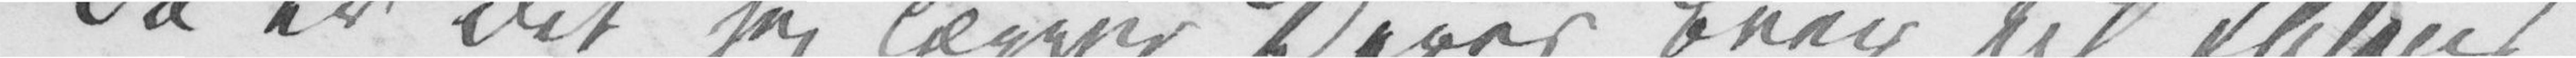Da er det jeg lægger Deres Brev til Ibsens
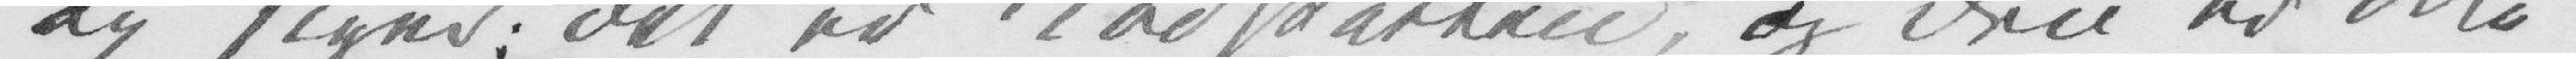og siger: det er Nødskatten, og den er ikke
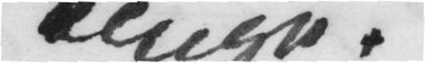ringe.
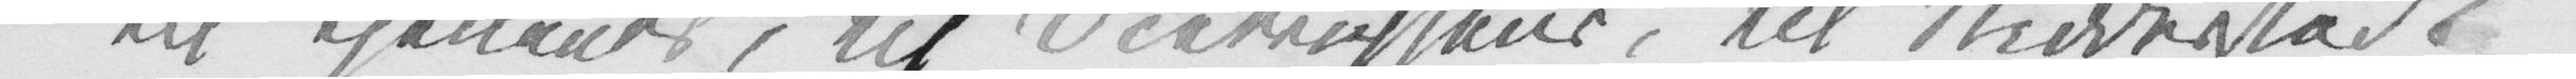til Kjellands, til Dietrichsons, til Ridderstads,
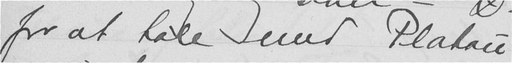for at tale med Platou
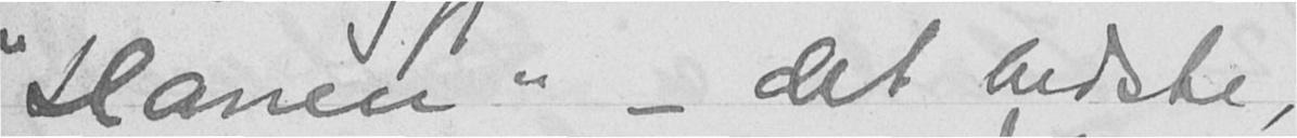"Hanen" - det bedste,
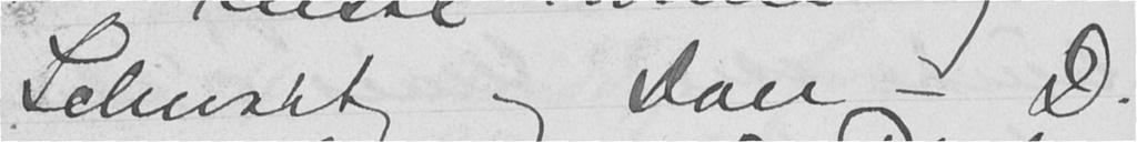Schwartz og Don - D.
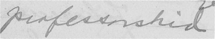professorstrid
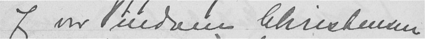Jeg var indom Christensen
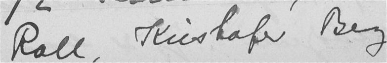Roll, Kristofer Berg
